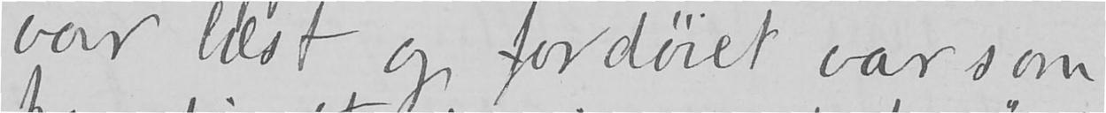var læst og fordøiet var som
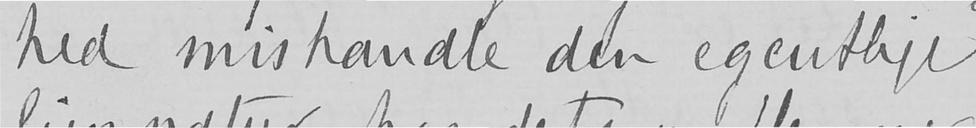hed mishandle den egentlige
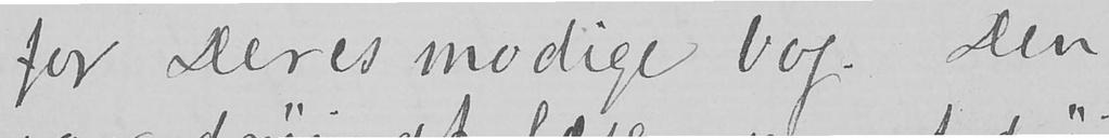for Deres modige bog. Den
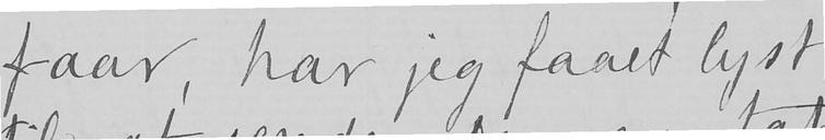faar, har jeg faaet lyst
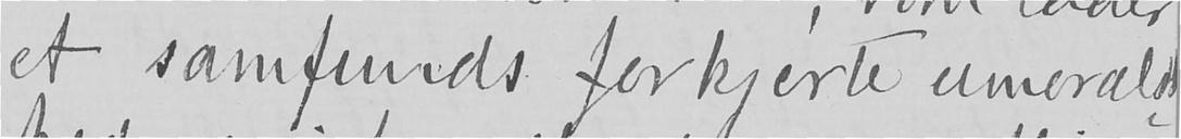et samfunds forkjerte umoralsk-
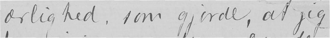ærlighed, som gjorde, at jeg
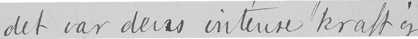det var dens intense kraft og
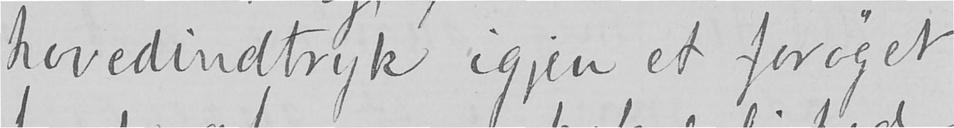hovedindtryk igjen et forøget
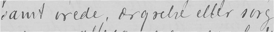samt vrede, ærgrelse eller sorg
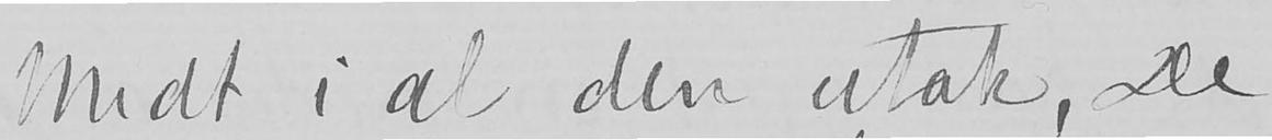Midt i al den utak, De
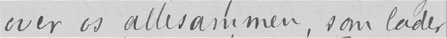over os allesammen, som lader
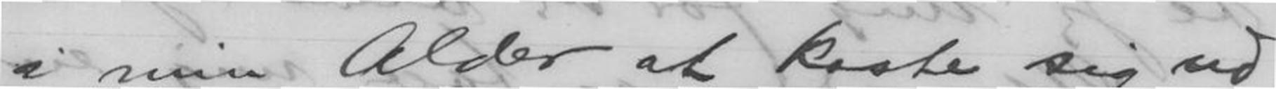i min Alder at kaste sig ud
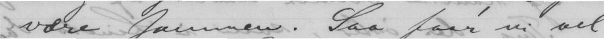være sammen. saa faar vi vel
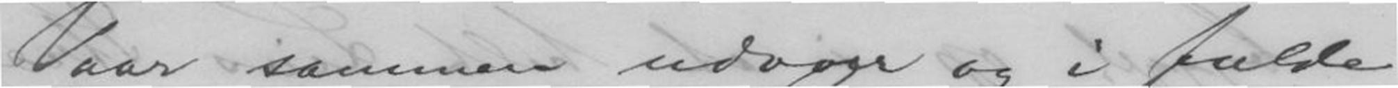Vaar sammen udover og i fulde
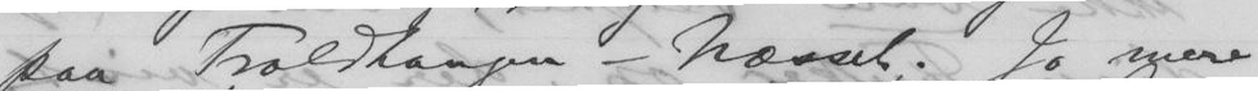paa Troldhaugen - Næsset. Jo mere
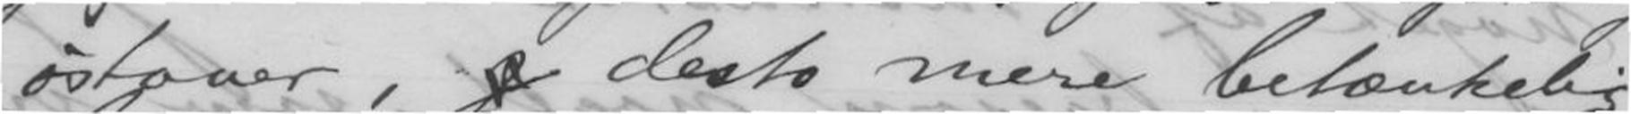østover, j desto mere betænkelig
